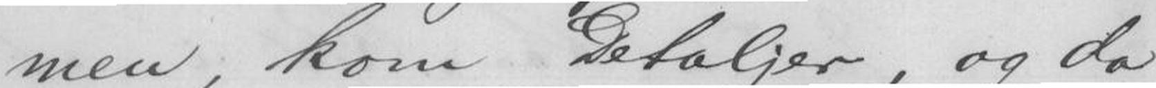men, kom Detaljer, og da
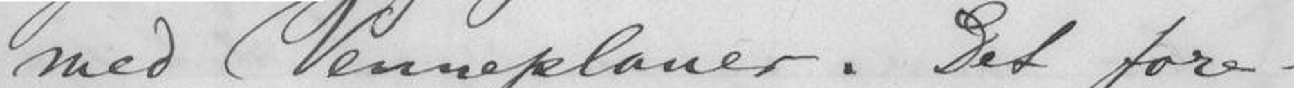med Venneplaner. Det fore-
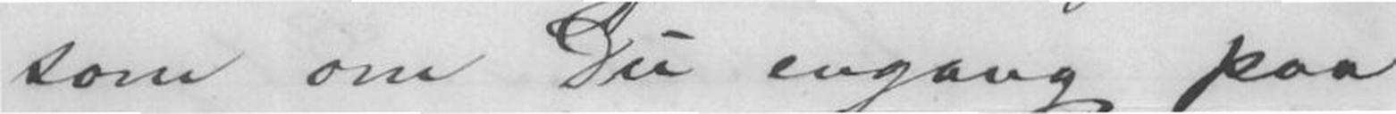som om Du engang paa
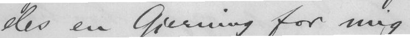des en Gjerning for mig
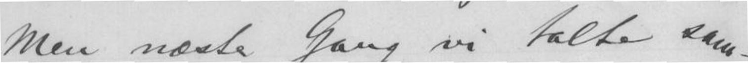Men næste Gang vi talte sam-
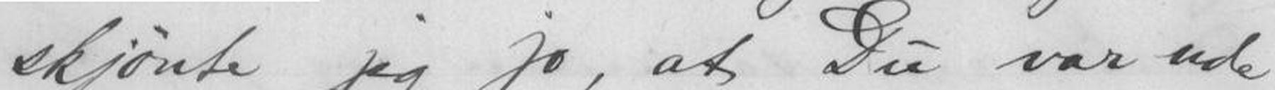skjønte jeg jo, at Du var ude
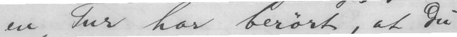en Tur har berørt, at Du
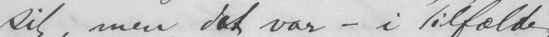sit, men det var - i Tilfældet,
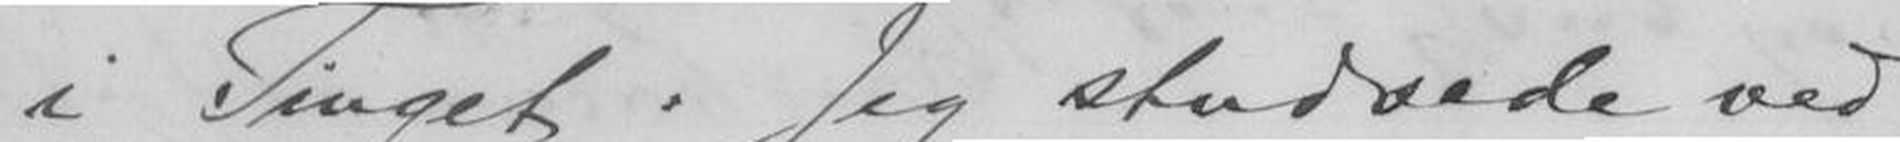i Tinget. Jeg studsede ved
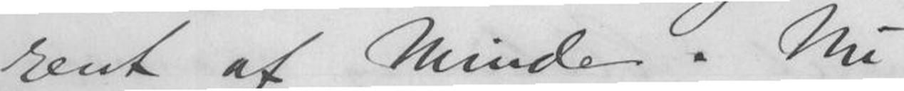rent af Minde. Nu,
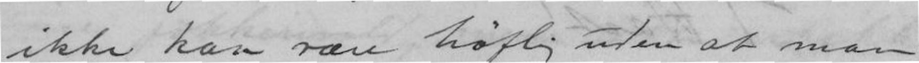ikke kan være høflig uden at man
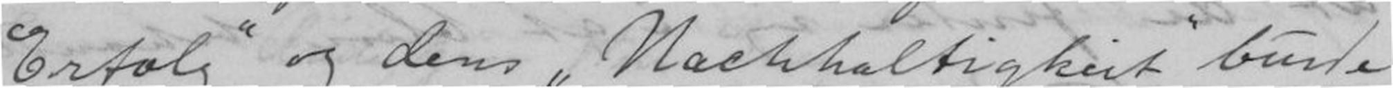"Erfolg" og dens "Nachhaltigkeit" burde
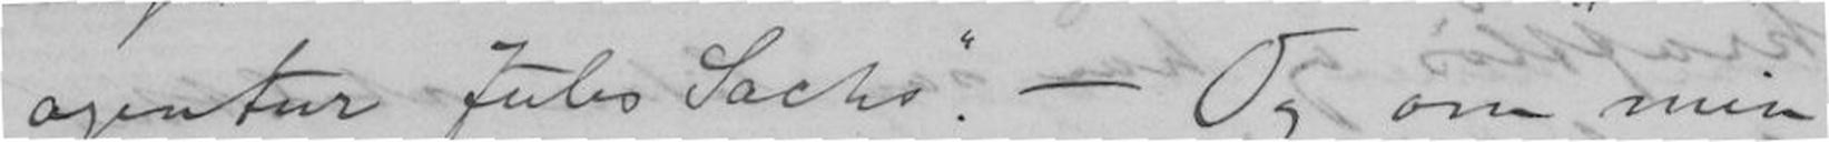agentur Jules Sachs". - Og om min
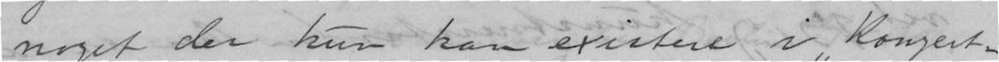noget der kun han existere i "Konzert-
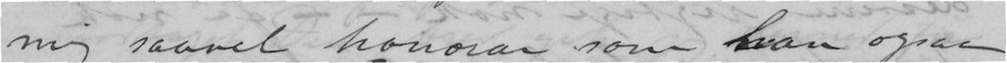mig saavel honorar som han ogsaa
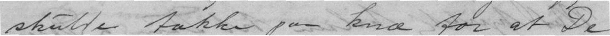skulde takke paa knæ for at De
